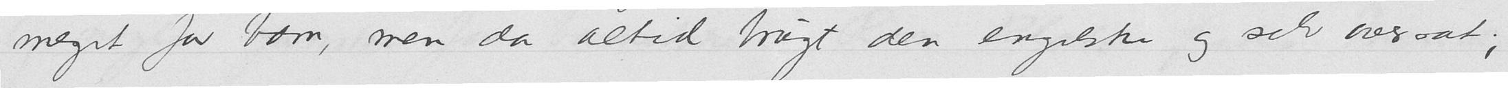meget for barn, men da altid brugt den engelske og selv oversat;
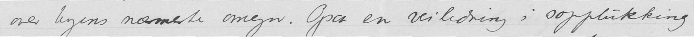over byens nærmeste omegn. Ogsaa en veiledning i sopplukking
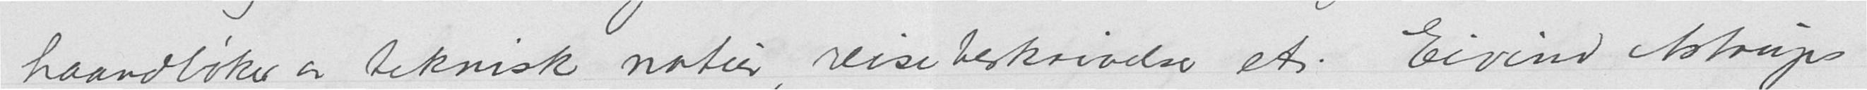haandbøker av teknisk natur, reisebeskrivelser etc. Eivind Astrups
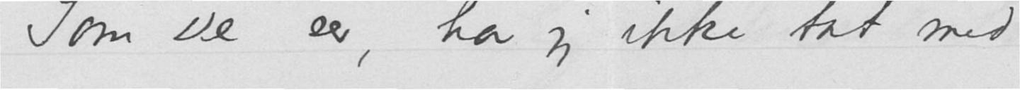Som De ser, har jeg ikke tat med
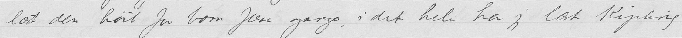læst den høit for barn flere gange, i det hele har jeg læst Kipling
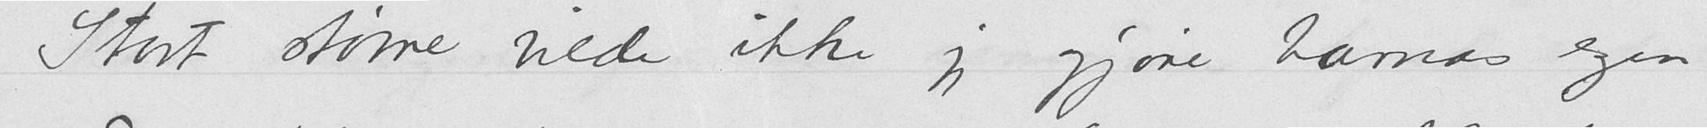Stort større vilde ikke jeg gjøre barnas egen
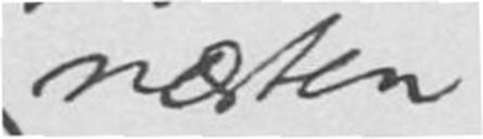næsten
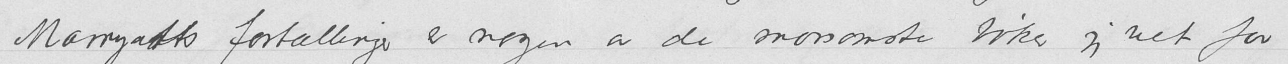Marryatts fortællinger er nogen av de morsomste bøker jeg vet for
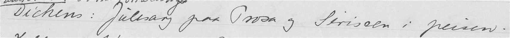Dickens: Julesang paa Prosa og Sirissen i peisen.
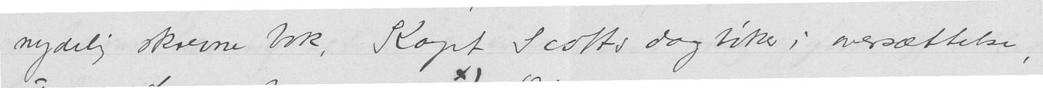nydelig skrevne bok, Kapt Scotts dagbøker i oversættelse,
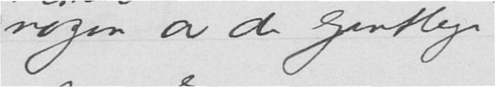nogen av de egentlige
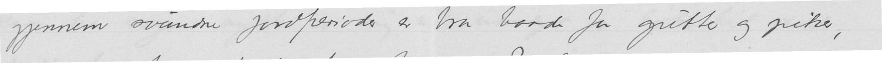gjennem svundne jordperioder er bra baade for gutter og piker,
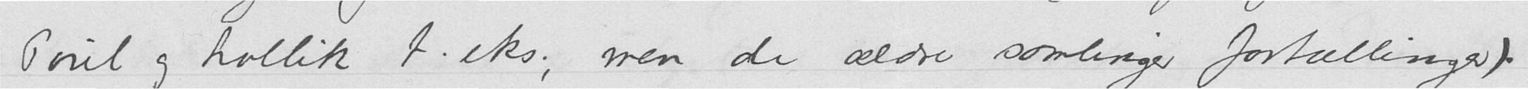Poul og Lollik f.eks., men de ældre samlinger fortællinger).
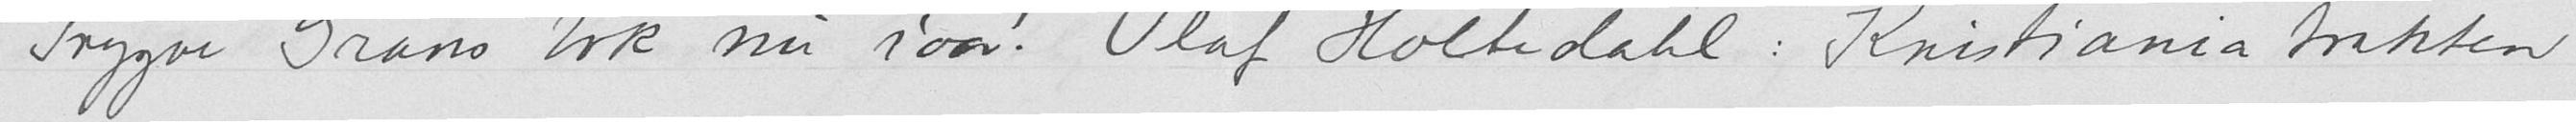Trygve Grans bok nu iaarx) Olaf Holtedahl: Kristiania trakten
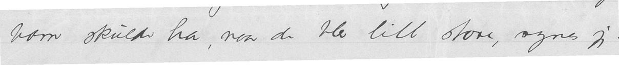barn skulde ha, naar de blev litt store, synes jeg.
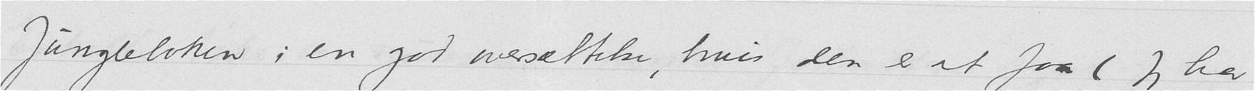Jungelboken i en god oversættelse, hvis den er at faa (Jeg har
